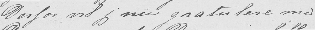Derfor vil jeg nu gratulere med
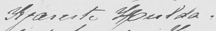Kjæreste Hulda.
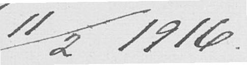11/2 1916.
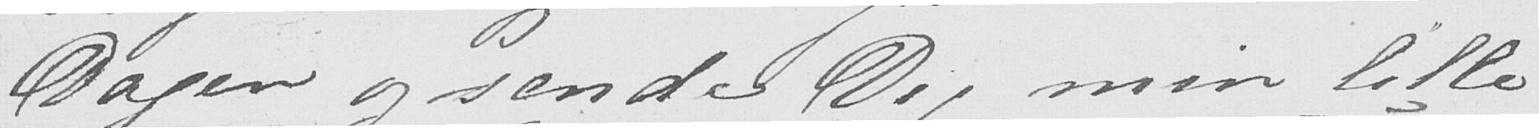Dagen og sende Dig min lille
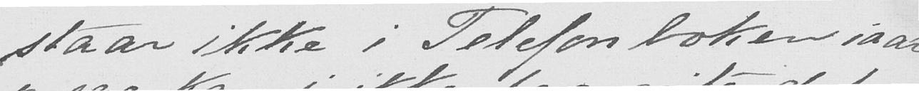staar ikke i Telefonboken iaar
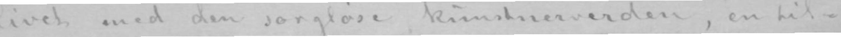livet med den sorgløse kunstnerverden, en til-
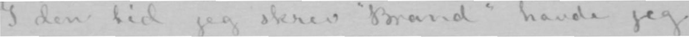I den tid jeg skrev «Brand» havde jeg
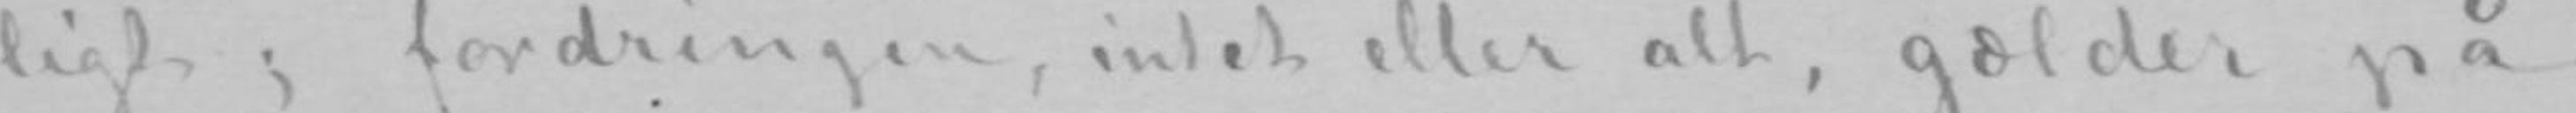ligt; fordringen, intet eller alt, gælder på
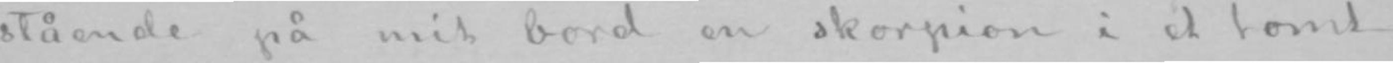stående på mit bord en skorpion i et tomt
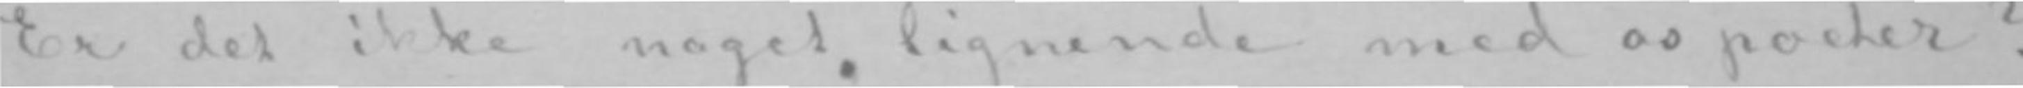Er det ikke noget lignende med os poeter?
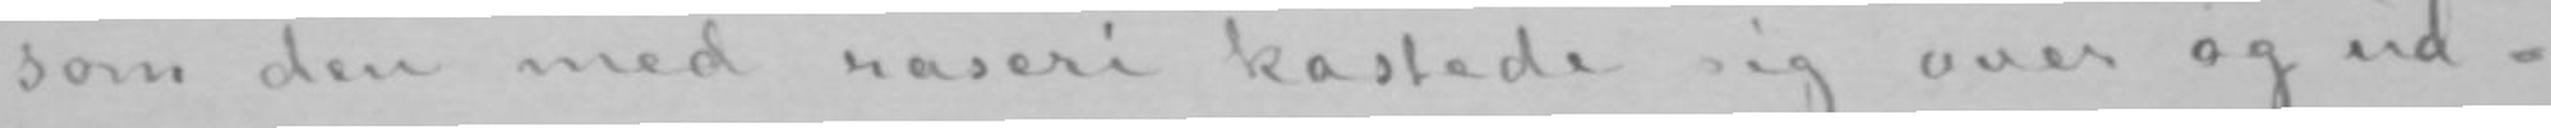som den med raseri kastede sig over og ud-
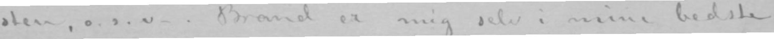sten, o. s. v-. Brand er mig selv i mine bedste
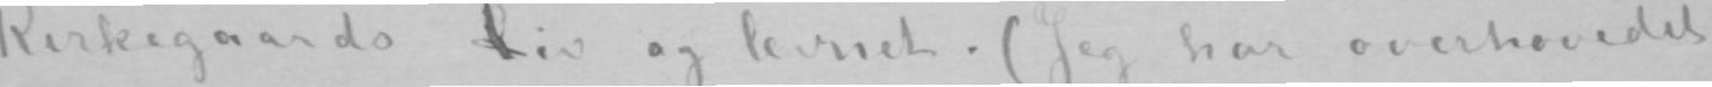Kirkegaards livL og levnet. (Jeg har overhovedet
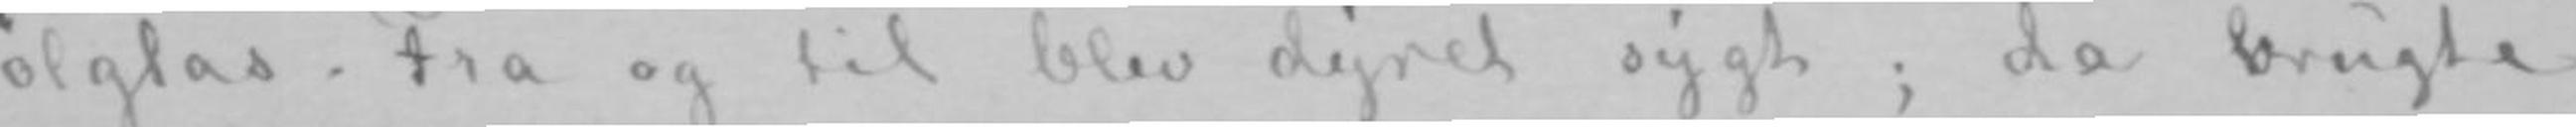ølglas. Fra og til blev dyret sygt; da brugte
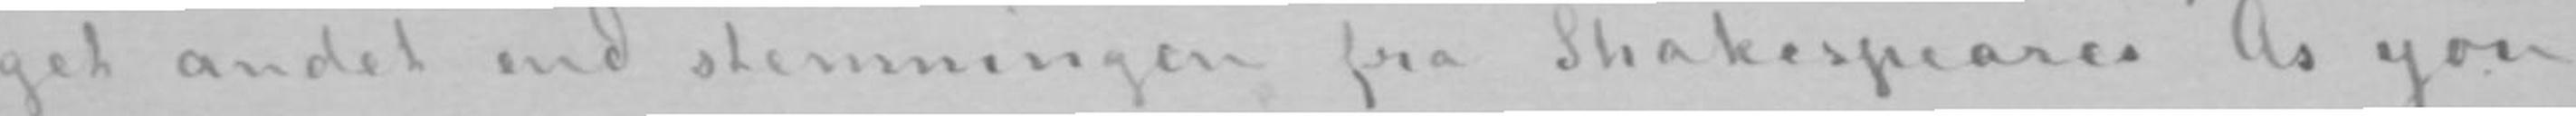get andet end stemningen fra Shakespeares «As you
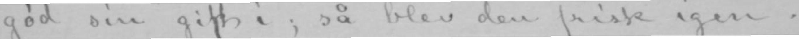gød sin gift i; så blev den frisk igen.
